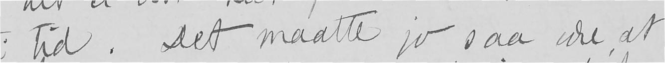tid. Det maatte jo saa være, at
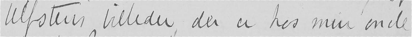Ulfstens billeder, der er hos min oncle
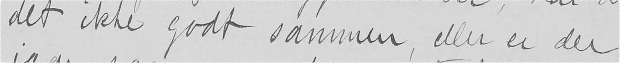det ikke godt sammen, eller er der
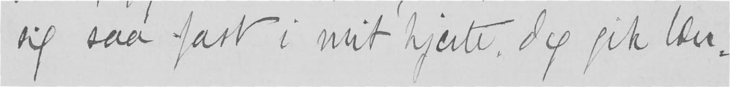sig saa fast i mit hjerte. Jeg gik læn-
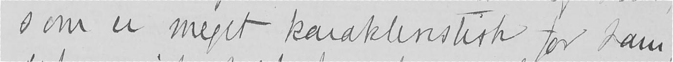som er meget karakteristisk for ham,
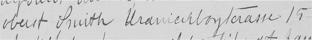oberst Smith Uranienborgterasse 15
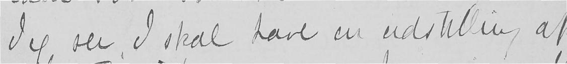Jeg ser, I skal have en udstilling af
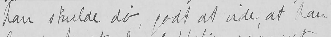han skulde dø, godt at vide at han
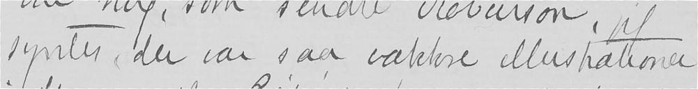syntes, der var saa vakkre illustrationer
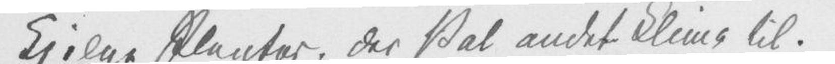kjelne Planter, der skal andet Klima til.
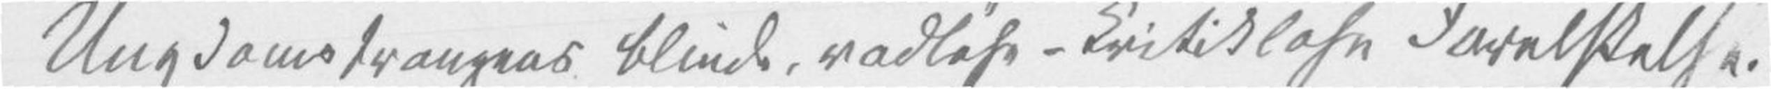Ungdomstrangens blinde, rodløse – kritikløse Forelskelse.
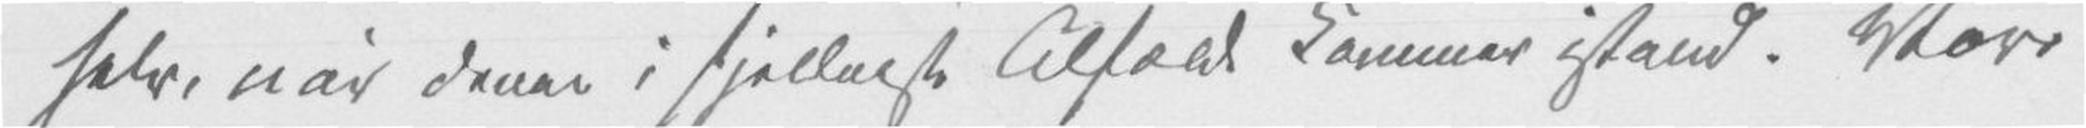selv, når denne i sjeldneste Tilfælde kommer istand. Vore
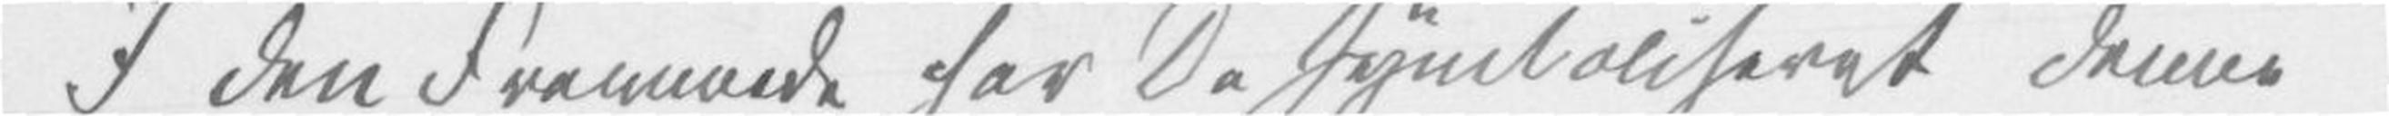I «den Fremmede» har De symboliseret denne
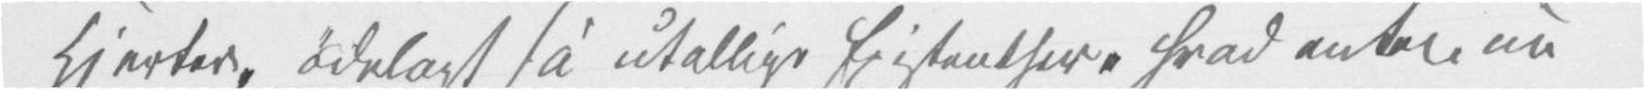Hjerter, ødelagt så utallige Existentser, hvad enten nu
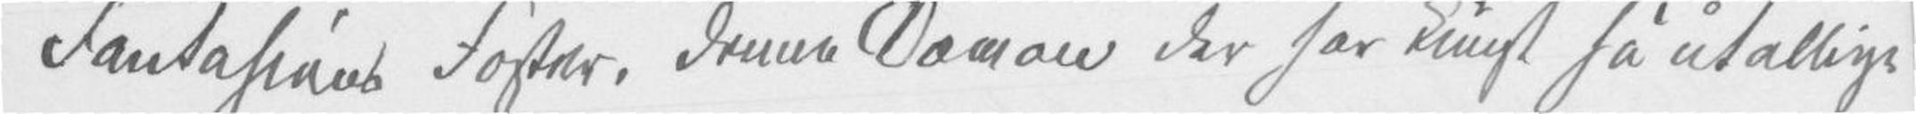Fantasiens Foster, denne Dæmon der har knust så utallige
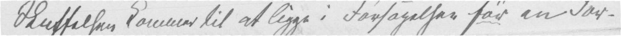Skuffelsen kommer til at ligge i Forsagelsen før en For¬
